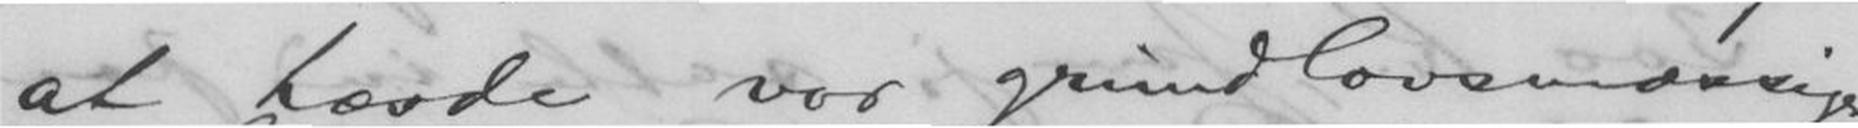at hævde vor grundlovsmæssige
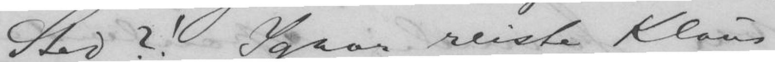Sted? Igaar reiste Klaus
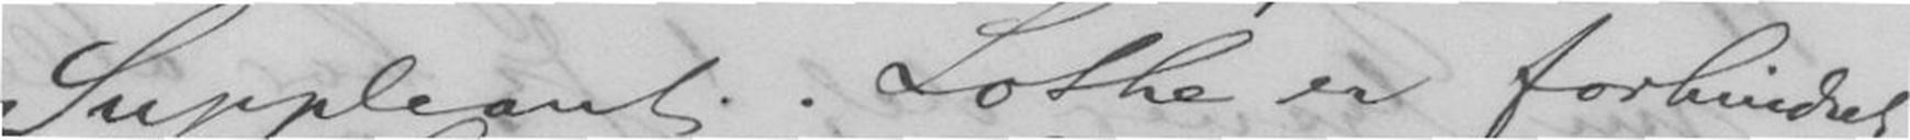Suppleant. Lothe er forhindret
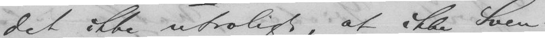det ikke utroligt, at ikke Sven-
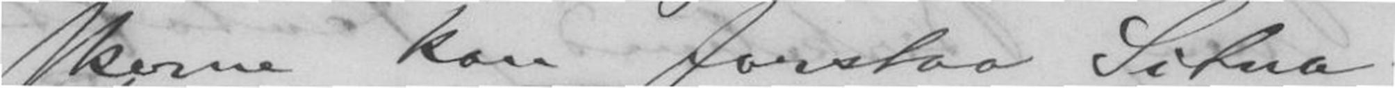skerne kan forstaa Situa-
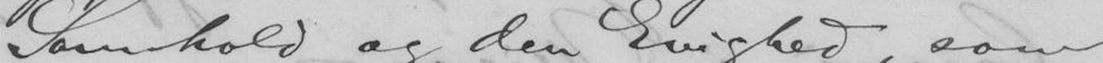Samhold og den Enighed, som
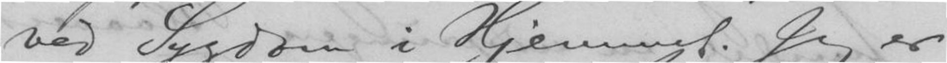ved Sygdom i Hjemmet. Jeg er
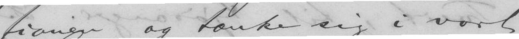tionen og tænke sig i vort
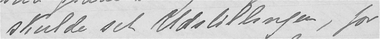skulde set Udstillingen, for
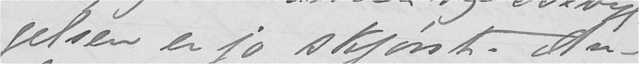gelsen er jo skjønt. An-
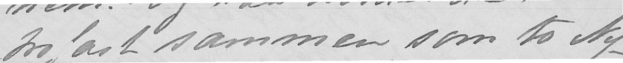trofast sammen, som to Ny-
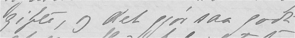gifte, og det gjør saa godt
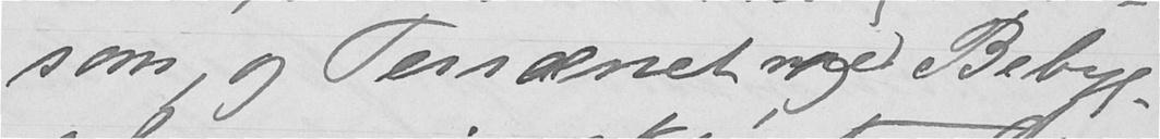som, og Terrænet med Bebyg-
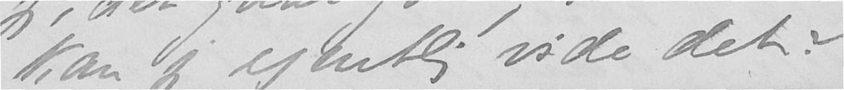kan jeg egentlig vide det?
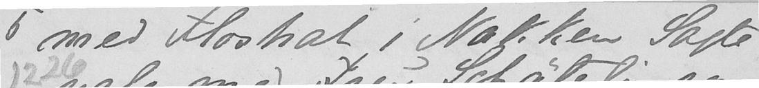med Floshat i Nakken Sagte
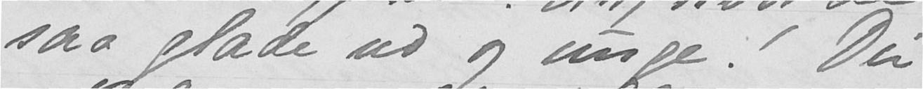saa glade ud og unge! Du
